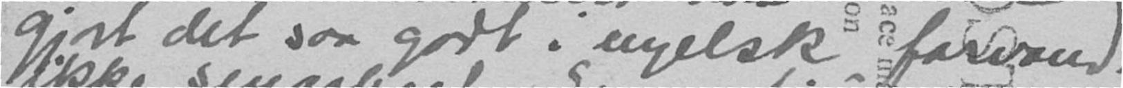gjort det saa godt i engelsk farvand -
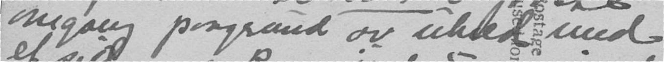omgang paagrund av uheld med
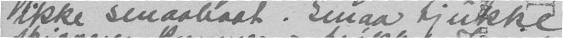ikke smaabaat. Smaa tjukke
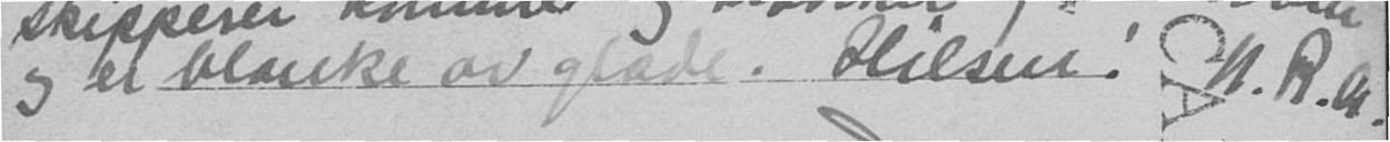og er blanke av glæde. Hilsen! N.R.A.
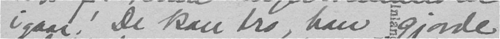i gaar! De kan tro, han gjorde
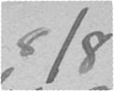8/8
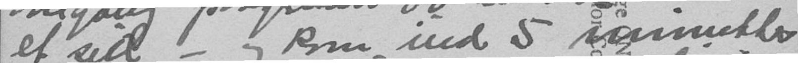et seil - og kom ind 5 minutter
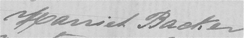Harriet Backer
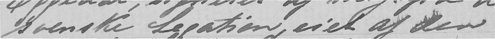svenske Legation eiet af den
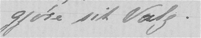gjøre sit Valg.
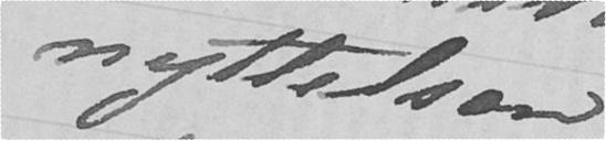nyttelsen
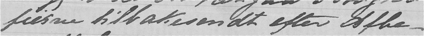fierne tilbakesendt efter Afbe-
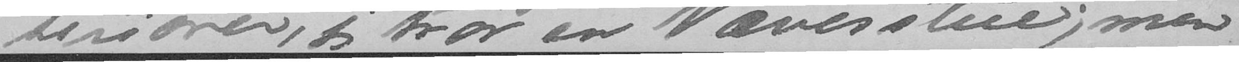teriører, jeg tror en Væverstue; men
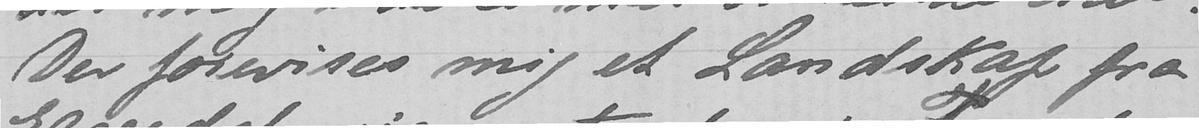Der forevises mig et Landskap fra
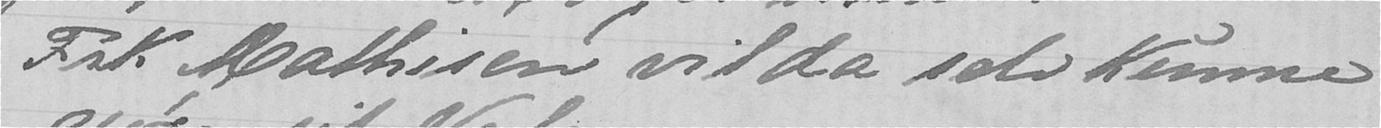Frk Mathisen vil da selv kunne
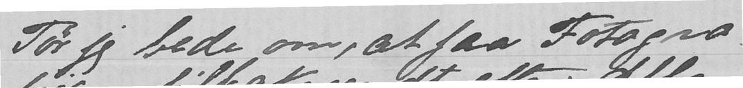Tør jeg bede om, at faa Fotogra-
